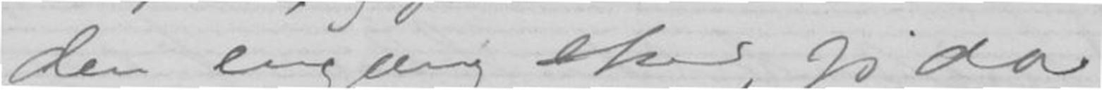der engang sker, jeg da
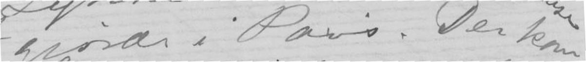gjorde i Paris. Der kom
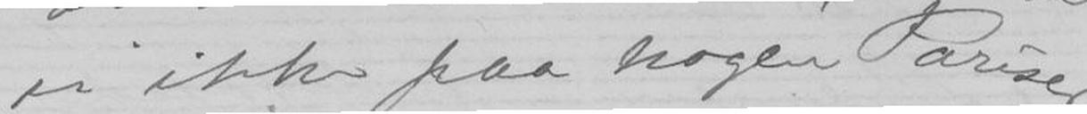vi ikke paa nogen Pariser
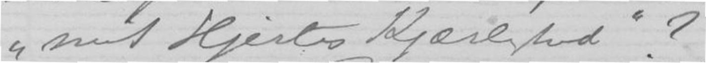"mit Hjertes Kjærlighed"?
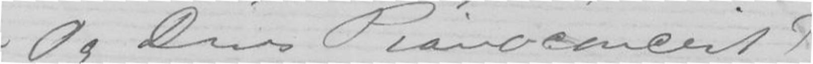Og Deres Pianoconcert?
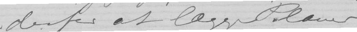derfor at lægge Planer,
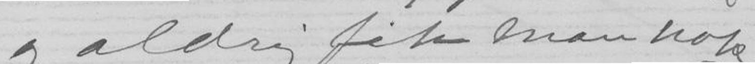og aldrig fik man nok
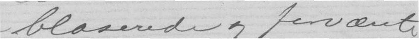blaserede og forvænte
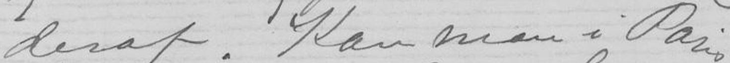deraf. Kan man i Paris
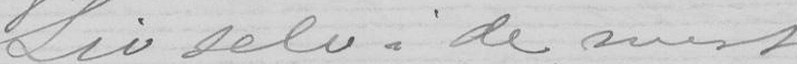Liv selv i de mest
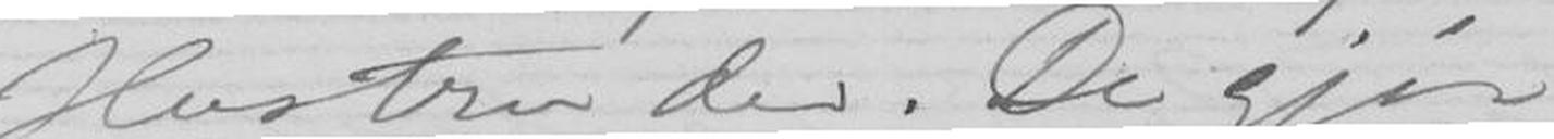Hustru der. De gjør
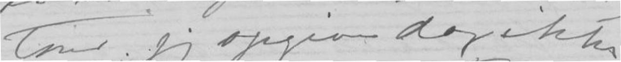tour, jeg opgive dog ikke
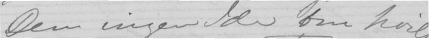Dem ingen Ide om hvilken
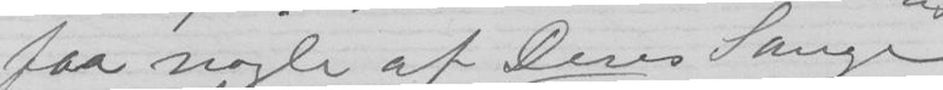faa nogle af Deres Sange
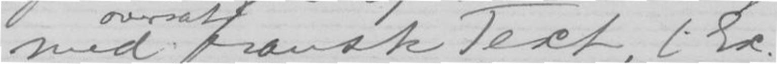med fransk Text, t Ex.
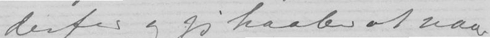derfor, og jeg haaber at naar
